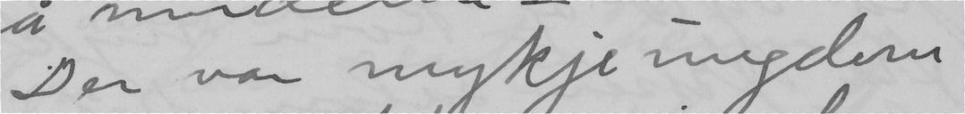Der var mykje ungdom
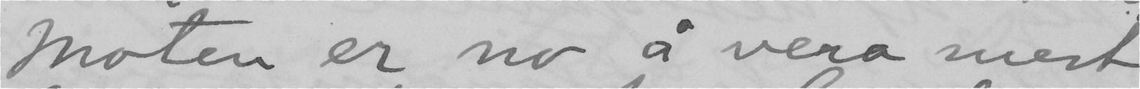Moten er no å vera mest
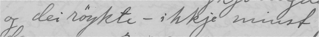og dei röykte – ikkje minst
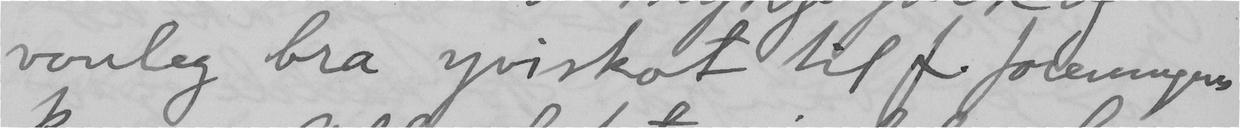vonleg bra yviskot til f.foreningens
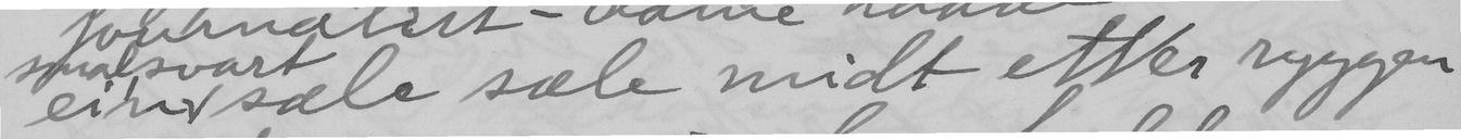ein smal svart svart sæle midt etter ryggen
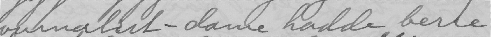formalist-dame hadde berre
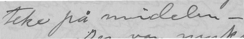teke på midelen –
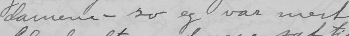damene – so eg var mest
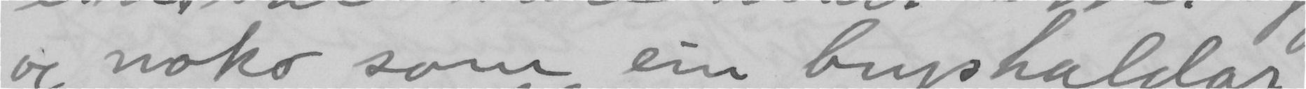og noko som ein bryshaldar
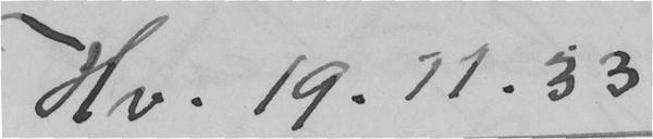Hv. 19.11.33
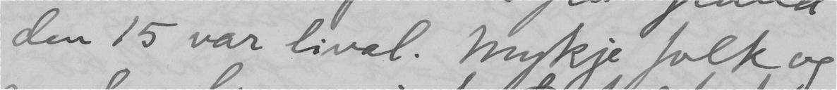den 15 var livat. Mykje folk og
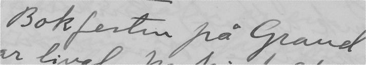Bokfesten på Grand
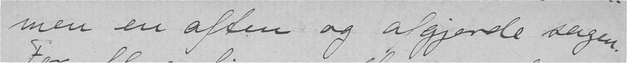men en aften og afgjorde sagen.
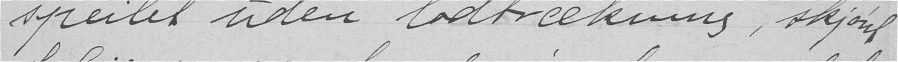speilet uden lodtrækning, skjønt
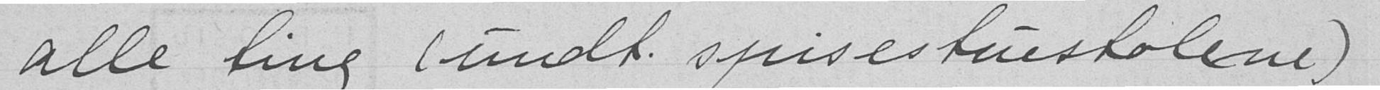alle ting (undt. spisestuestolene)
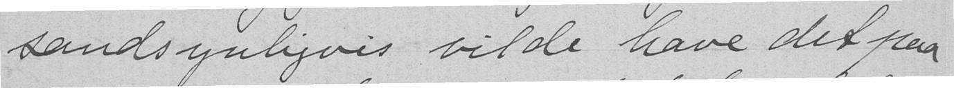sandsynligvis vilde have det paa
